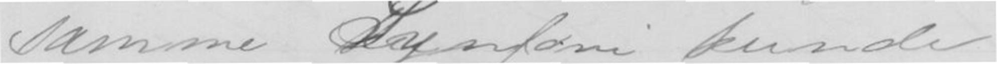samme Symfoni kunde
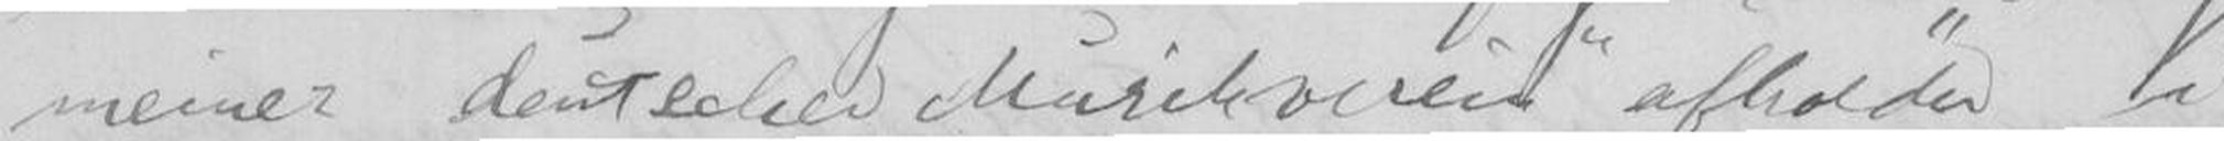meiner deutscher Musikverein afholder i
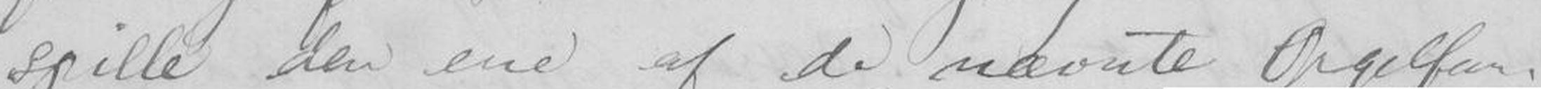spille den ene af de nævnte Orgelfan-
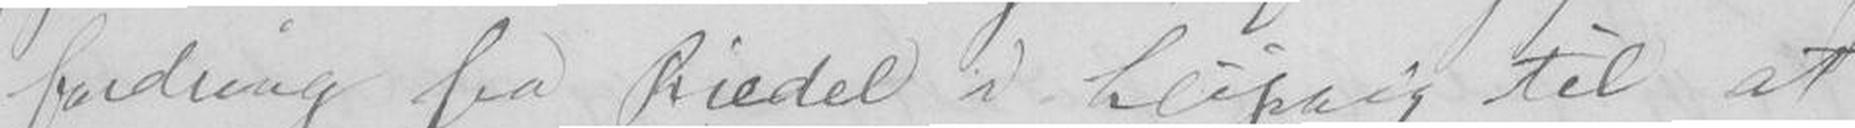fordring fra Riedel i Leipzig til at
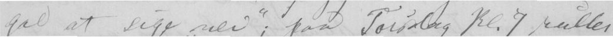god at sige nei; paa Torsdag Kl. 7 ruller
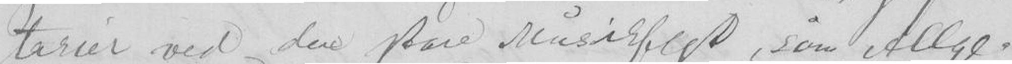tasier ved den store Musikfest, som Allge-
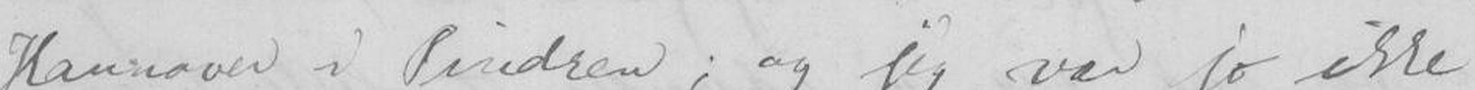Hannover i Pindsen; og jeg var jo ikke
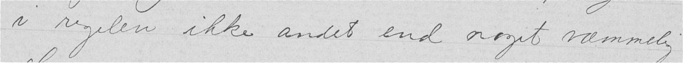i regelen ikke andet end noget væmmelig
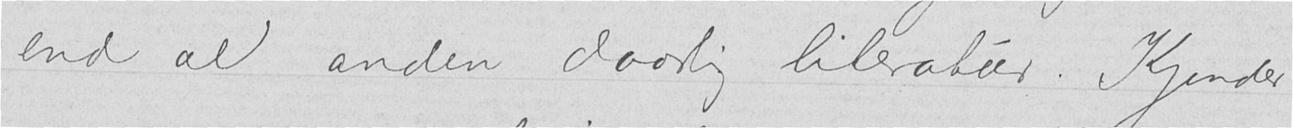end al anden daarlig literatur. Kjender
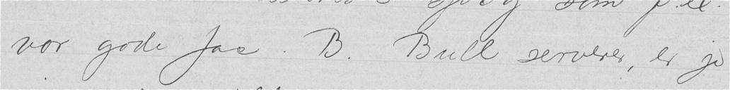vor gode Jac. B. Bull serverer, er jo
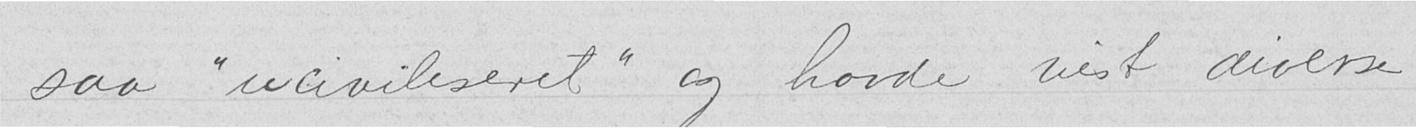saa "uciviliseret" og havde vist diverse
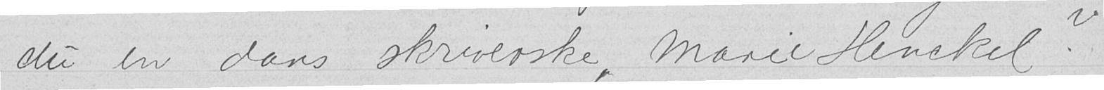du en dansk skriverske, Marie Henckel?
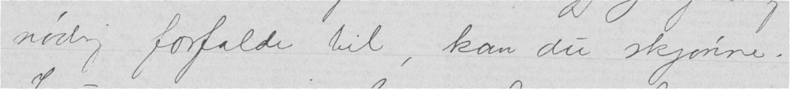nødig forfalde til, kan du skjønne.
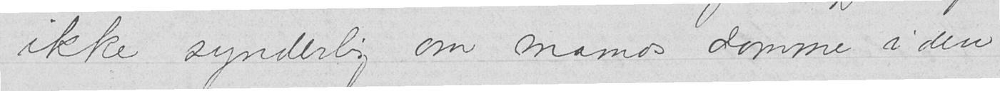ikke synderlig om mamas domme i den
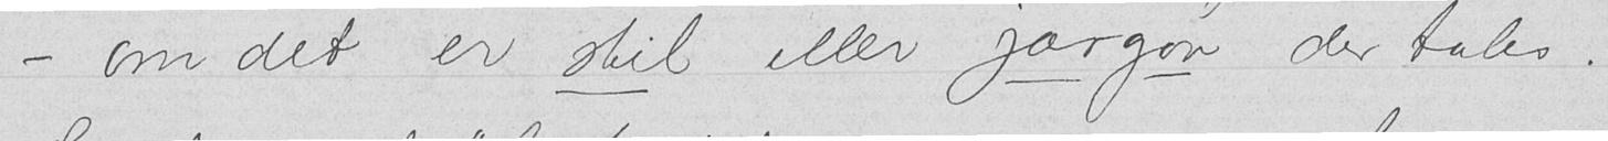- om det er stil eller jargon der tales.
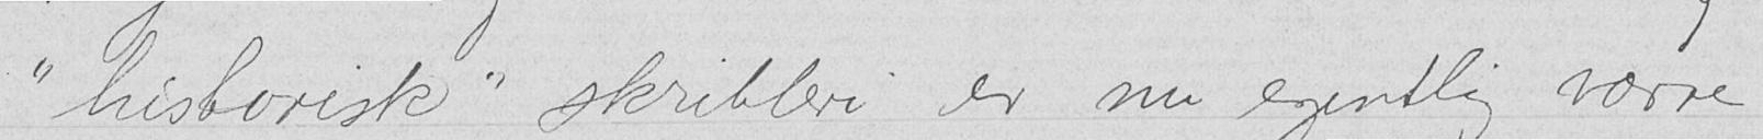"historisk" skribleri er nu egentlig værre
